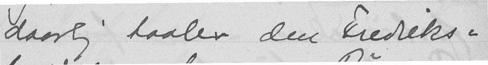daarlig taaler den Fredriks-
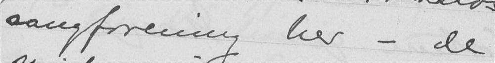sangforening her - de
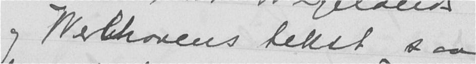og Welhavens tekst saa
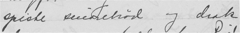spiste smørebrød og drak
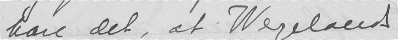bare det, at Wergelands
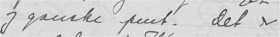og ganske pent. Det er
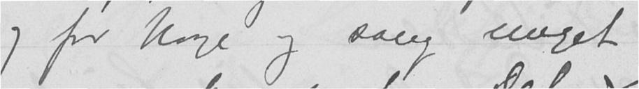og for Norge og sang meget
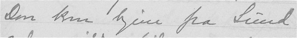Don kom hjem fra Sund
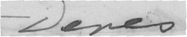Deres
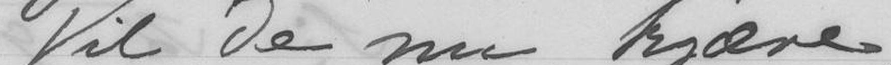Vil De nu kjære,
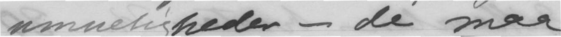umueligheder - de maa
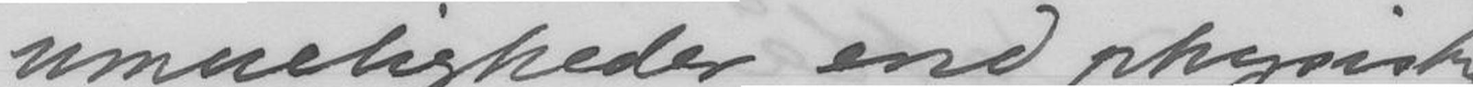umueligheder end physiske
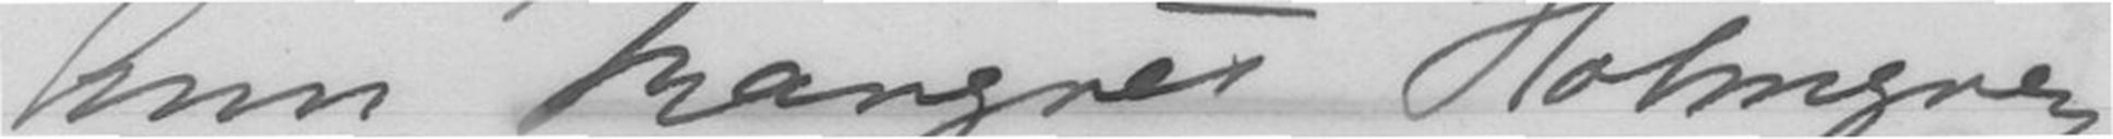Ann Magret Holmgren
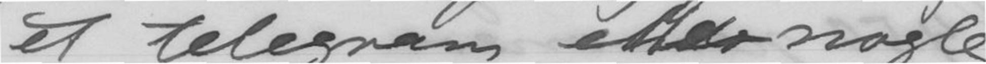et telegram eller nogle
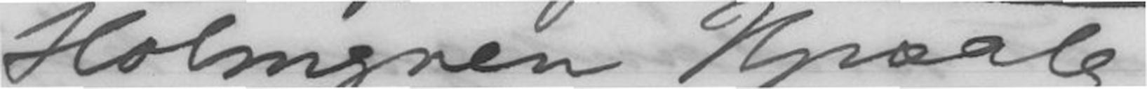Holmgren Upsala.
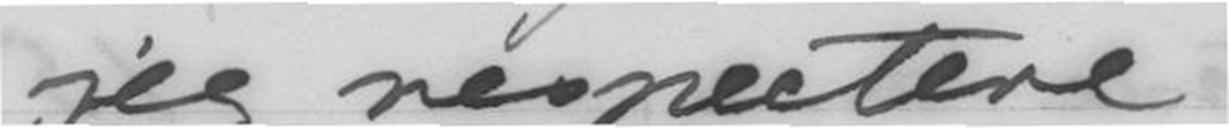jeg respectere!
